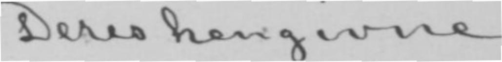Deres hengivne
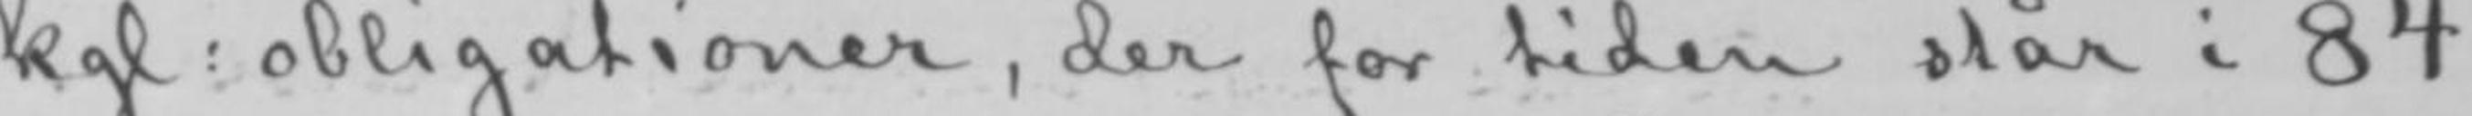kgl: obligationer, der for tiden står i 84
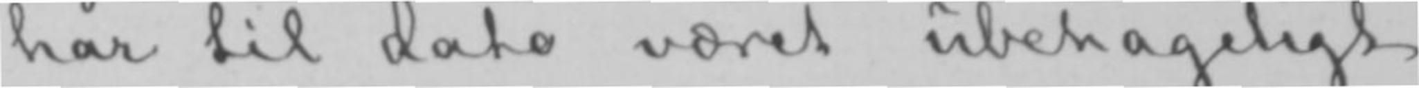har til dato været ubehageligt.
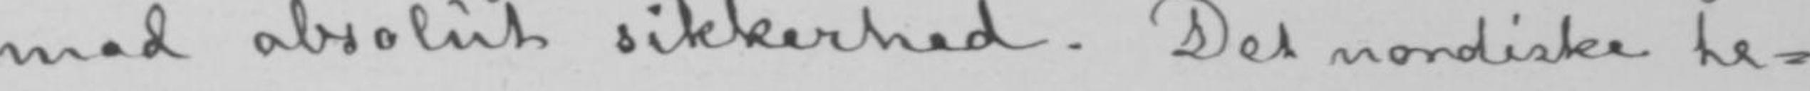mod absolut sikkerhed. Det nordiske te-
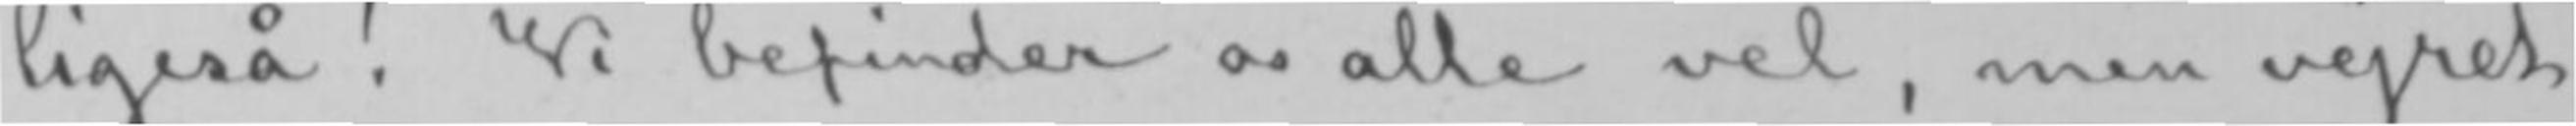ligeså! Vi befinder os alle vel, men vejret
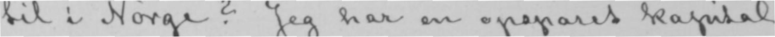til i Norge? Jeg har en opsparet kapital
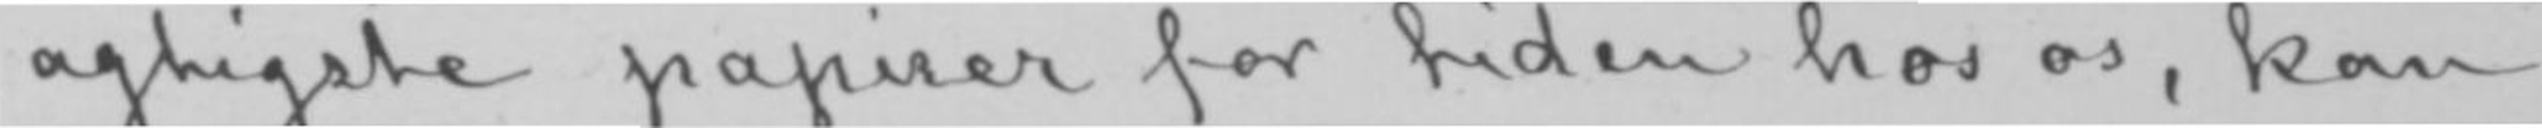agtigste papirer for tiden hos os, kan
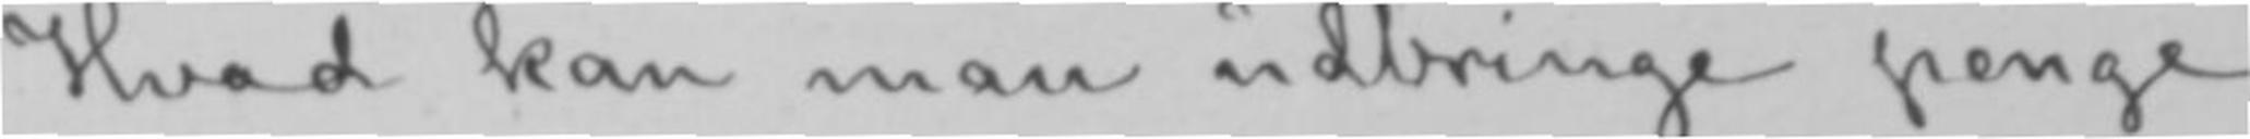Hvad kan man udbringe penge
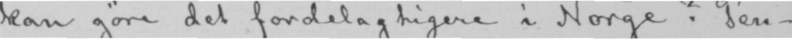kan gøre det fordelagtigere i Norge? Pen-
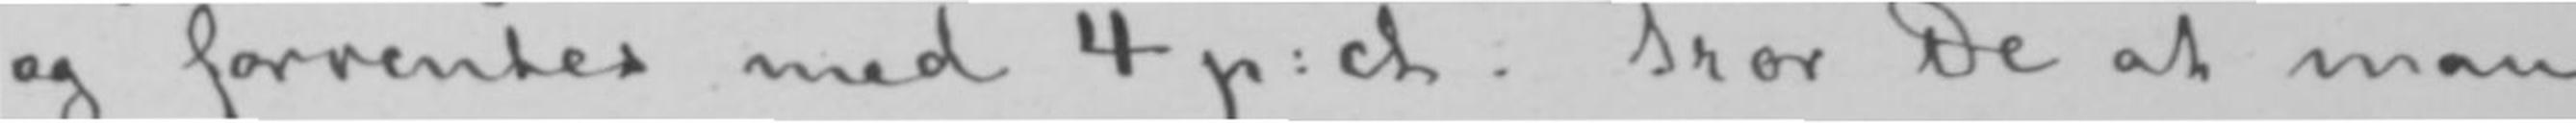og forrentes med 4 p:ct. Tror De at man
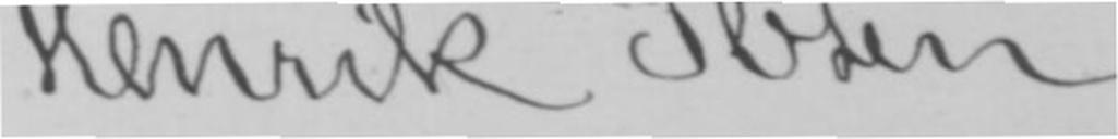Henrik Ibsen.
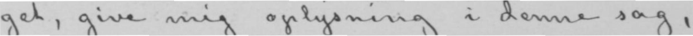get, give mig oplysning i denne sag,
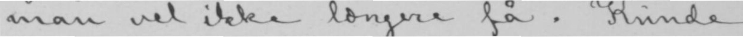man vel ikke længere få. Kunde
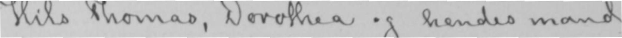Hils Thomas, Dorothea og hendes mand
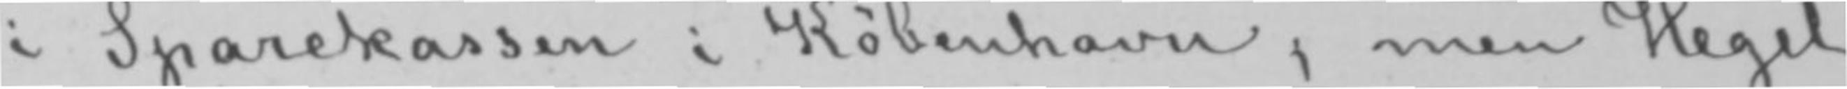i Sparekassen i København; men Hegel
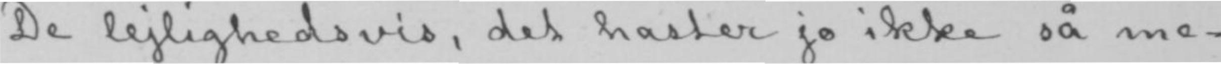De lejlighedsvis, det haster jo ikke så me-
